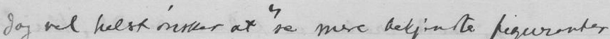dog vel helst ønskes at" se mere bekjendte figuranter
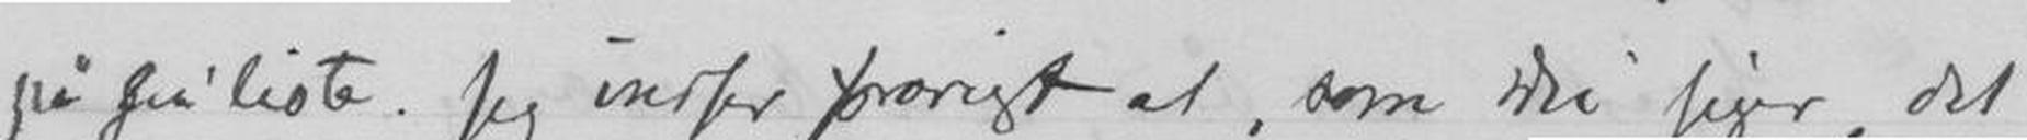på sin liste. Jeg indser forøvrigt at, som Du siger, det
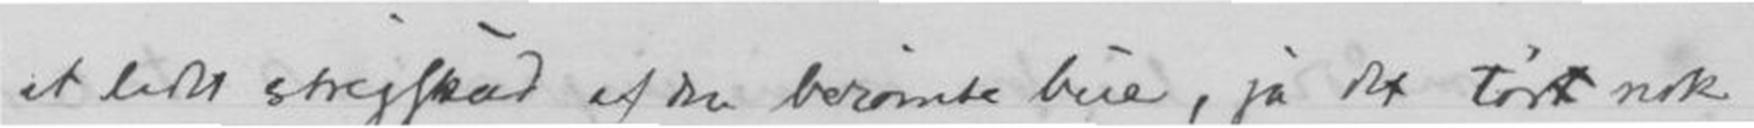et lidet strigskud af den berømte bue, ja det tør nok
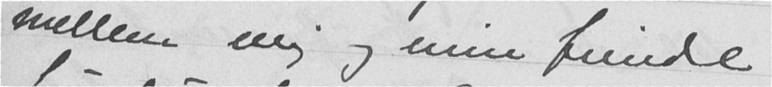mellem mig og min fiende
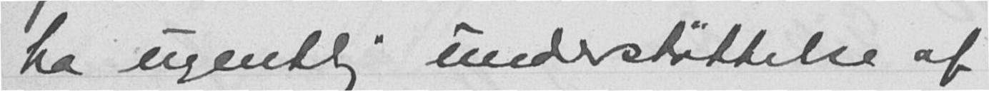ha ugentlig understøttelse af
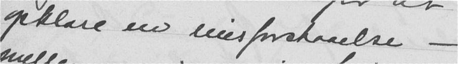opklare en misforstaaelse -
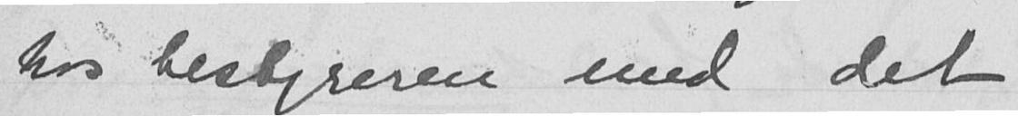hos bestyreren med det
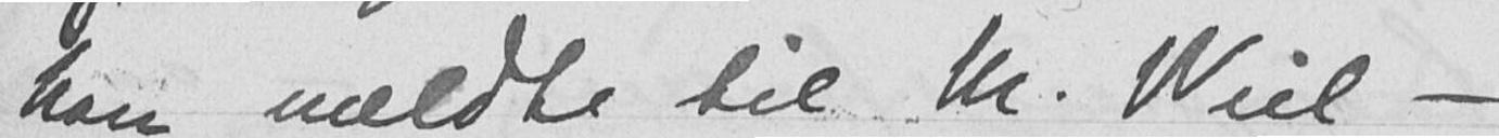han meldte til M. Wiel -
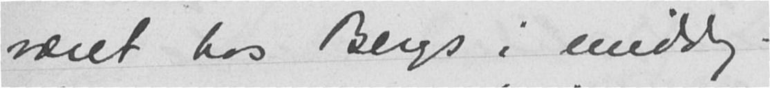været hos Bergs i middag.
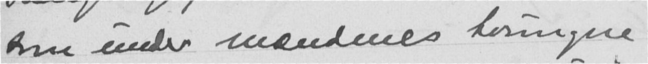som under mændenes tvungne
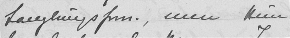Saugbrugsforn., men kun
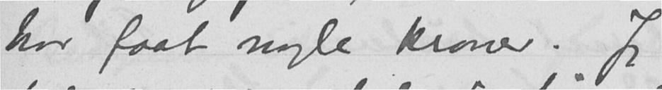har faat nogle kroner. Jeg
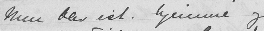Men blev ist. hjemme og
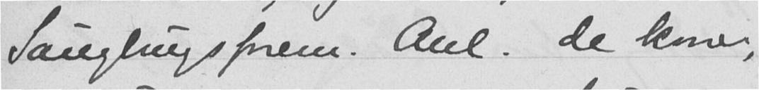Saugbrugsforene. Anl. de koner,
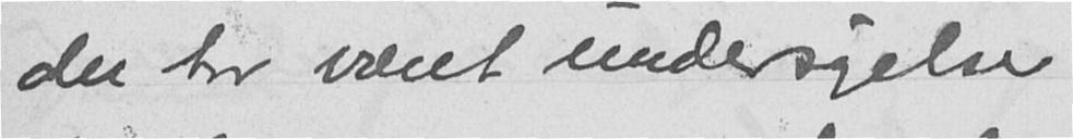der har været undersøgelser
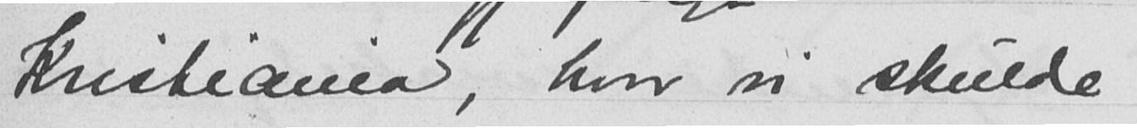Kristiania, hvor vi skulde
